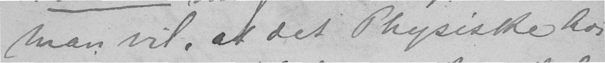man vil, at det Physiske hos
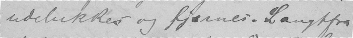udelukkes og fjernes. Langtfra
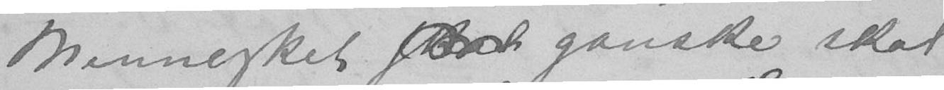Mennesket ganske skal
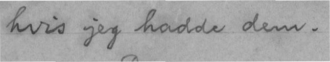hvis jeg hadde dem.
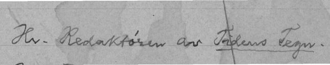Hr. Redaktøren av Tidens Tegn.
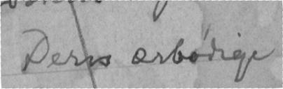Deres ærbødige
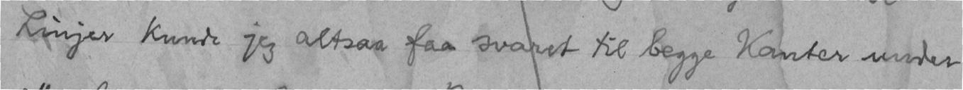Linjer kunde jeg altsaa faa svaret til begge Kanter under
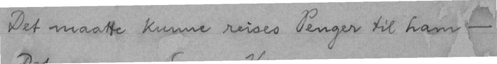Det maatte kunne reises Penger til ham -
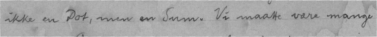ikke en Dot, men en Sum. Vi maatte være mange
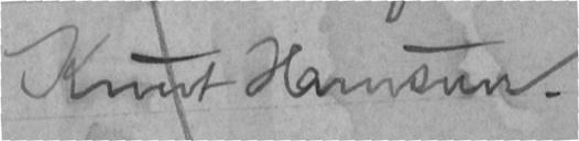Knut Hamsun.
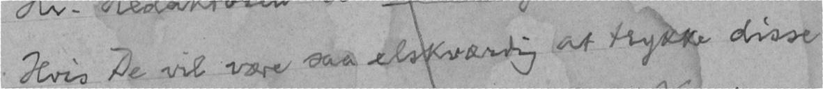Hvis De vil være saa elskværdig at trykke disse
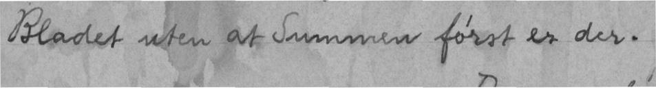Bladet uten at Summen først er der.
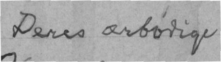Deres ærbødige
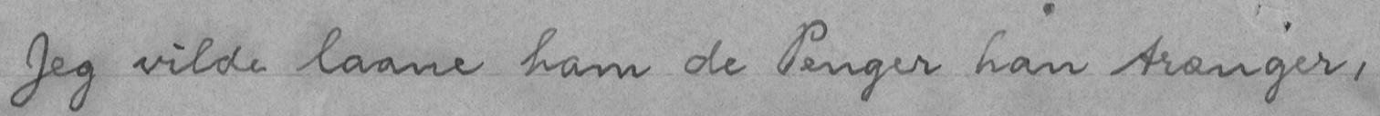Jeg vilde laane ham de Penger han trænger,
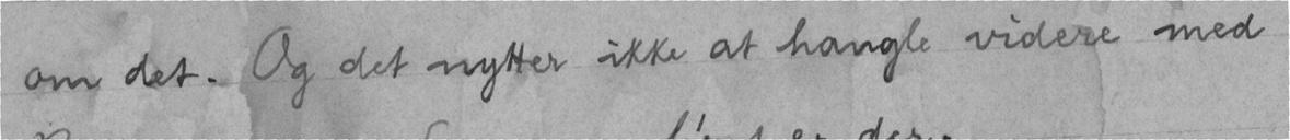om det. Og det nytter ikke at hangle videre med
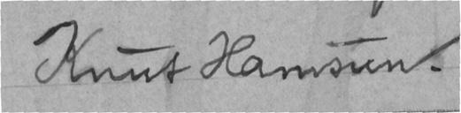Knut Hamsun.
